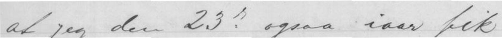at jeg den 23de ogsaa iaar fik
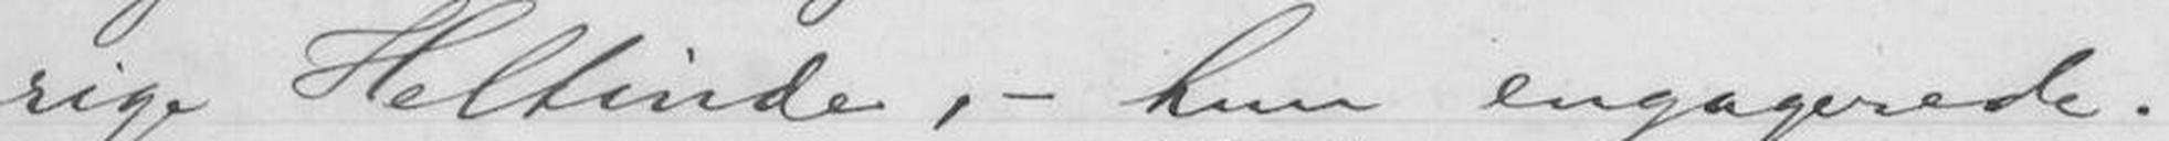rige Heltinde, - hun engagerede.
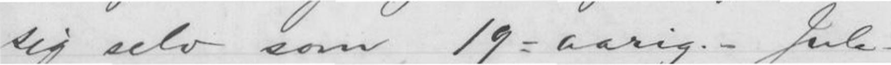sig selv som 19-aarig. - Jule-
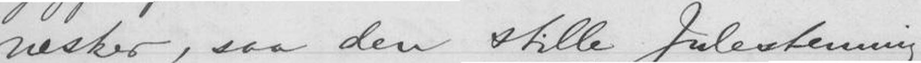nesker, saa den stille Julestemning
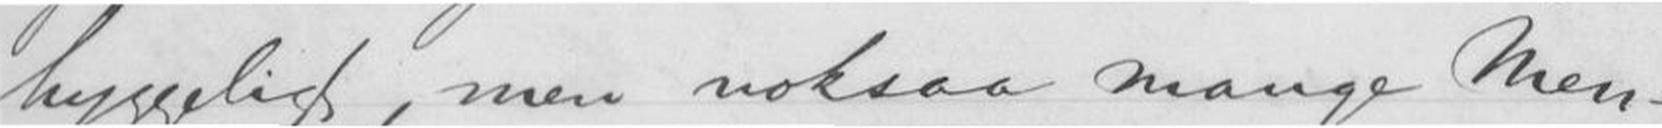hyggeligt, men noksaa mange Men-
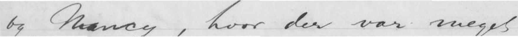og Nancy, hvor der var meget
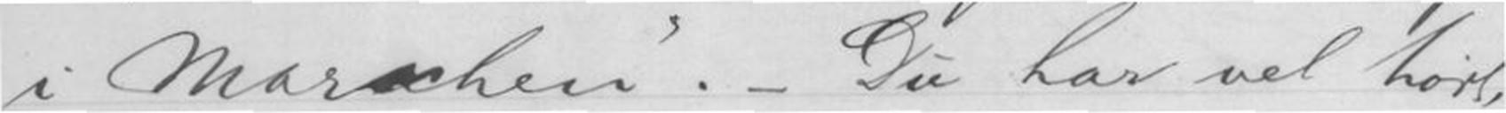i Marschen". - Du har vel hørt,
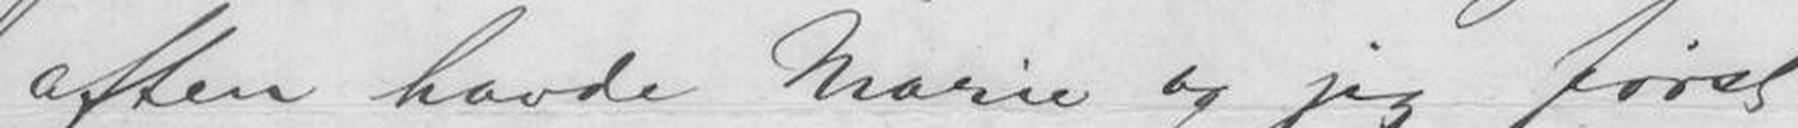aften havde Marie og jeg først
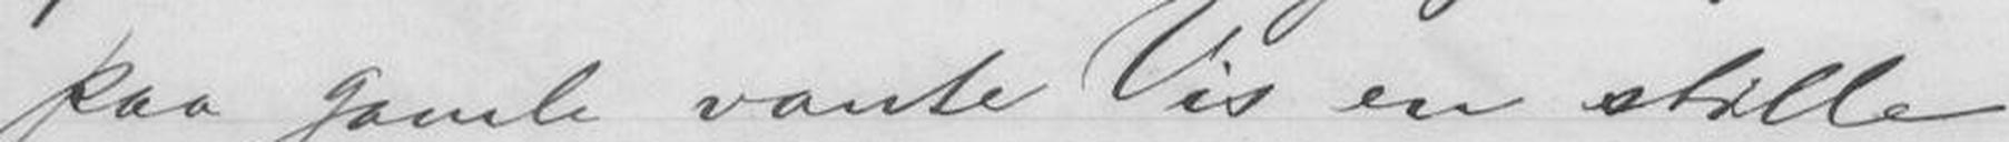paa gamle vante Vis en stille
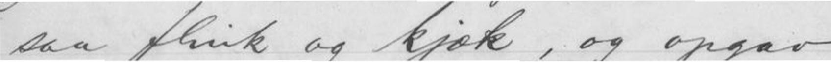saa flink og kjæk, og opgav
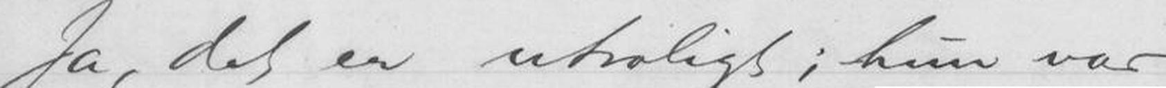Ja, det er utroligt; hun var
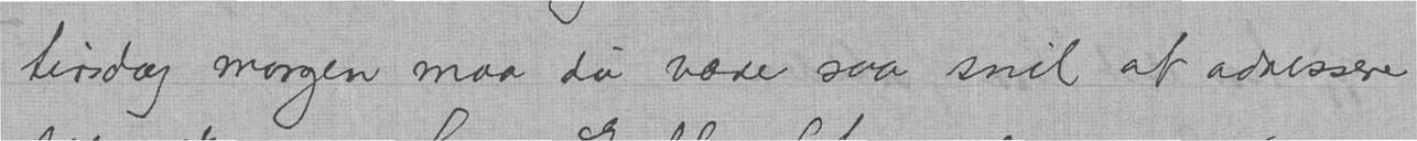tirsdag morgen maa du være saa snil at adressere
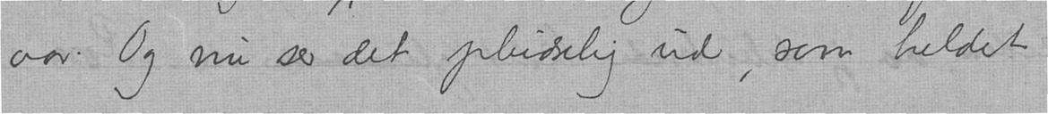aar. Og nu ser det pludselig ud, som heldet
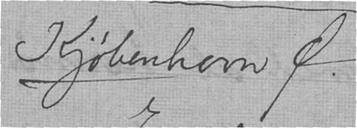Kjøbenhavn Ø.
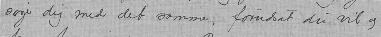søger dig med det samme, forudsat du vil og
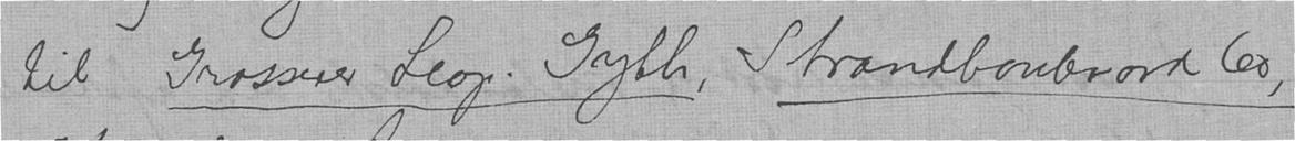til Grosserer Leop. Gyth, Strandboulevard 60,
